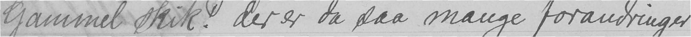Gammel skik! der er da saa mange forandringer
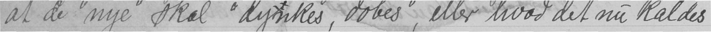at de "nye" skal "dynkes, døbes", eller hvad det nu kaldes
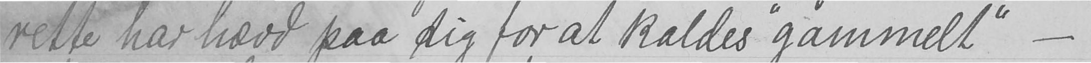rette har hævd paa sig for at kaldes "gammelt" -
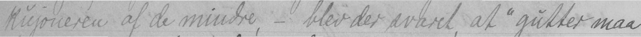kujoueren af de mindre, - blev der svaret, at gutter maa
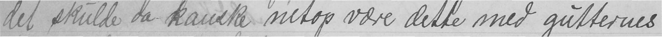det skulde da kanske netop være dette med gutternes
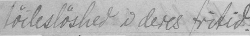tøilesløshed i deres fritid.
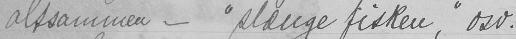altsammen - "slænge fisken," osv.
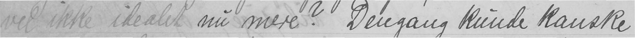vel ikke idealet nu mere Dengang kunde kanske
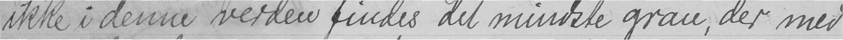ikke i denne verden findes det mindste gran, der med
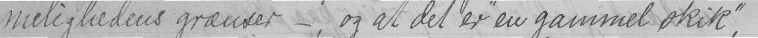melighedens grænser -, og at det er "en gammel skik,"
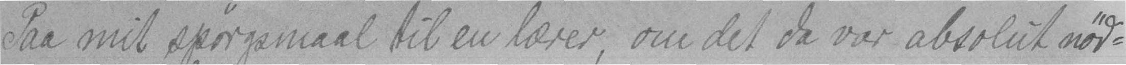Paa mit spørgsmaal til en lærer, om det da var absolut nød-
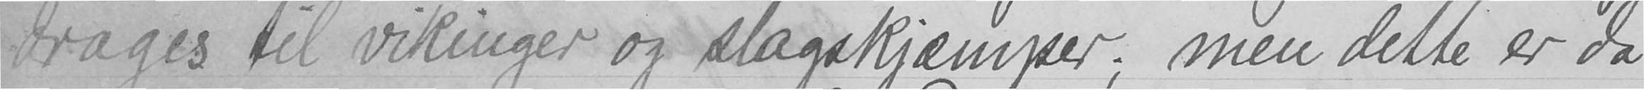drages til vikinger og slagskjæmper; men dette er da
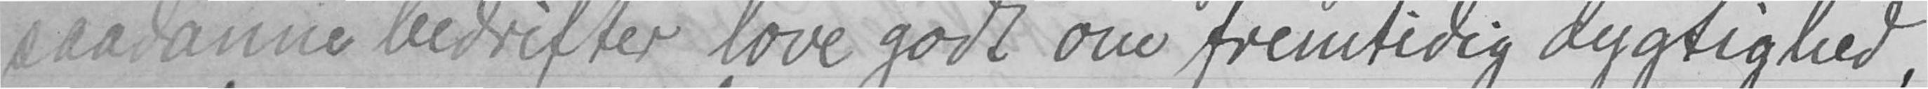saadanne bedrifter love godt om fremtidig dygtighed,
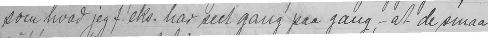som hvad jeg f. eks. har seet gang paa gang, - at de smaa
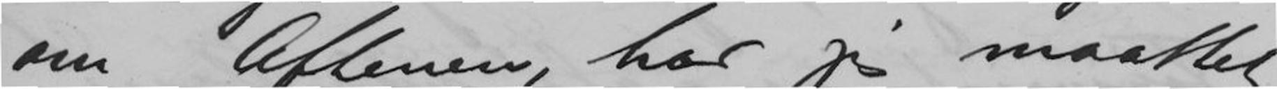om Aftenen, har jeg maattet
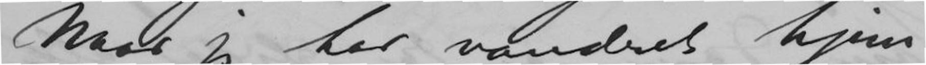Naar jeg har vandret hjem
